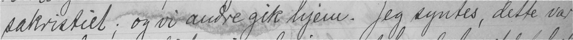sakristiet; og vi andre gik hjem. Jeg syntes, dette var
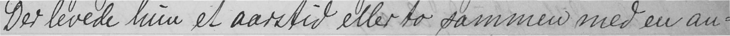Der levede hun et aarstid eller to sammen med en an-
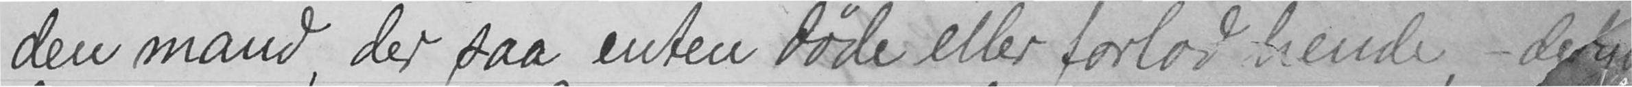den mand, der saa enten døde eller forlod hende, - det
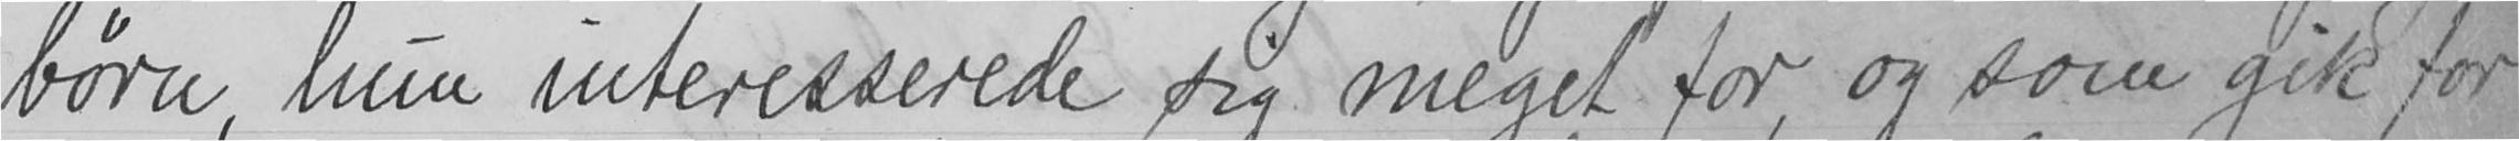børn, hun interesserede sig meget for, og som gik for
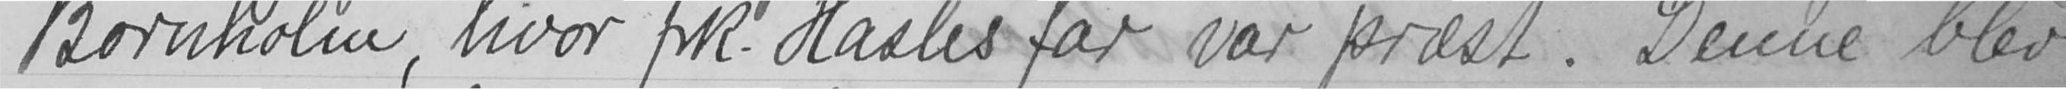Bornholm, hvor frk. Hasles far var præst. Denne blev
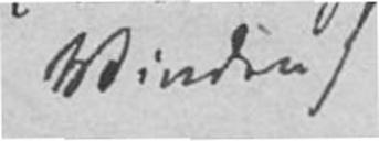Vinden)
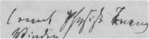(reent Physisk Tvang
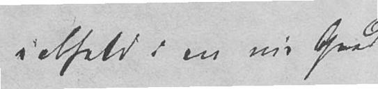ialfald i en vis Grad
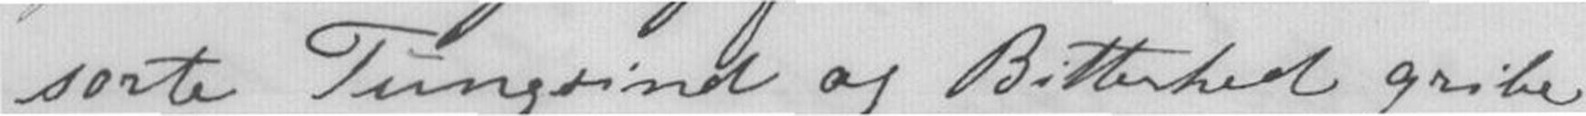sorte Tungsind og Bitterhed gribe
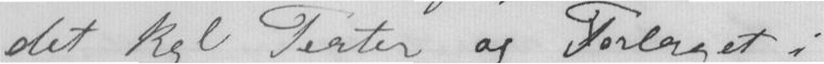det kgl Teater og Forlaget i
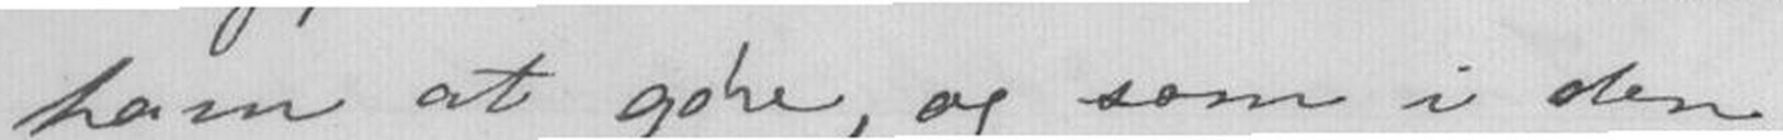ham at gøre, og som i den
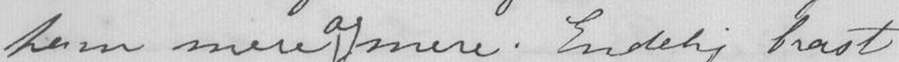ham mere og mere. Endelig brast
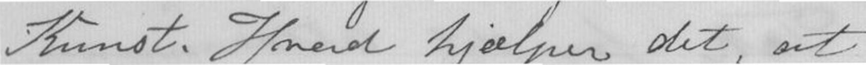Kunst. Hvad hjælper det, at
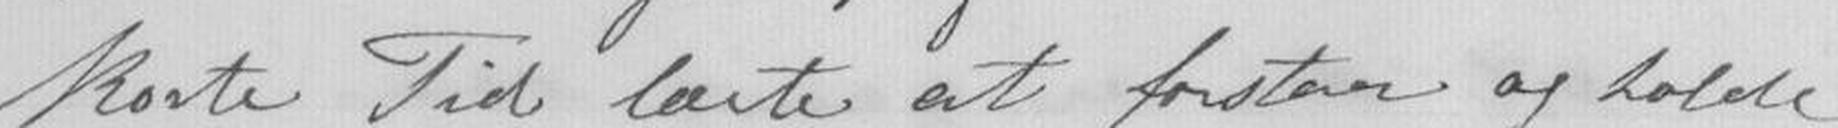korte Tid lærte at forstaa og holde
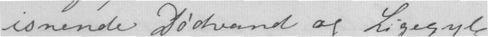isnende Dødvand og Ligesgyl-
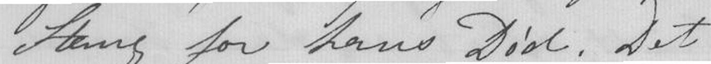Stang for hans Død. Det
